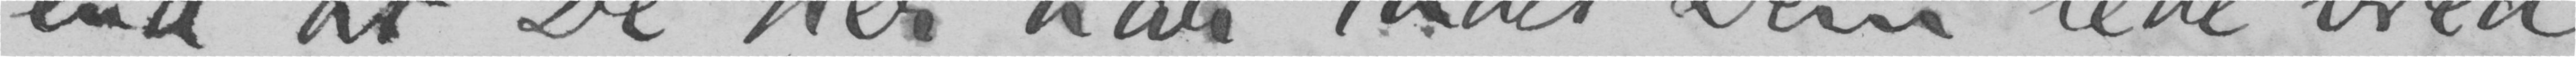end at De her har ladet Dem lede vild
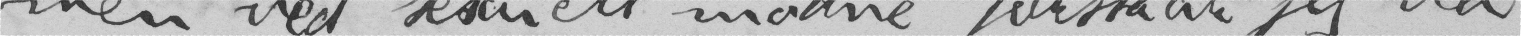men ved sexuelt modne forstaar jeg da
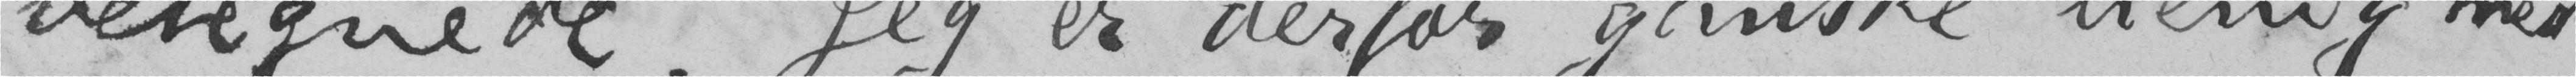betegnede.  Jeg er derfor ganske uenig med
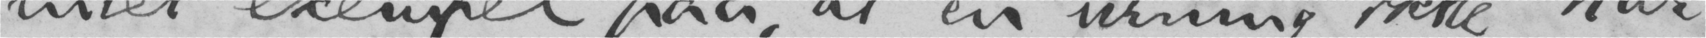intet exempel paa, at en urning ikke har
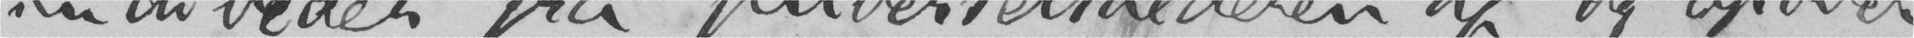individer fra pubertetsalderen af og opover,
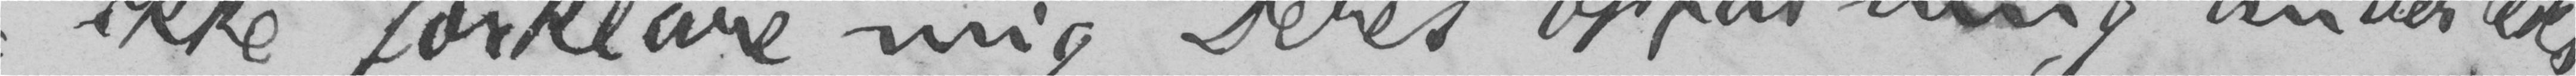ikke forklare mig Deres opfatning anderledes,
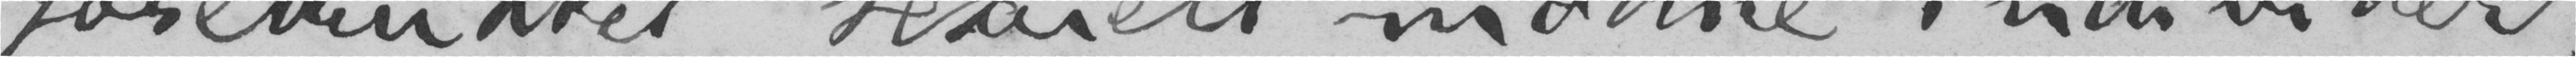foretrukket sexuelt modne individer;
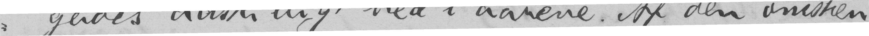gaaes adskilligt ned i aarene. Af den omstæn-
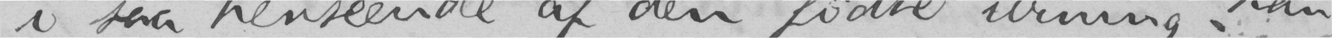i saa henseende af den fødte urning kan
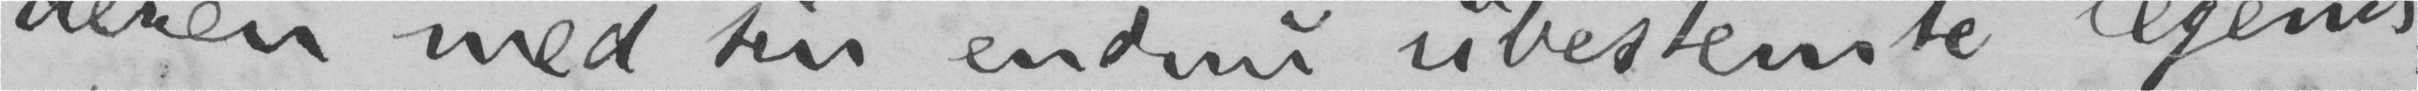deren med sin endnu ubestemte legems-
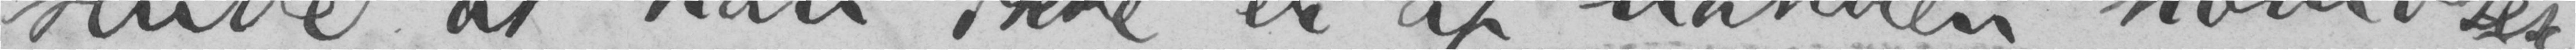slutte, at han ikke er af naturen homosex-
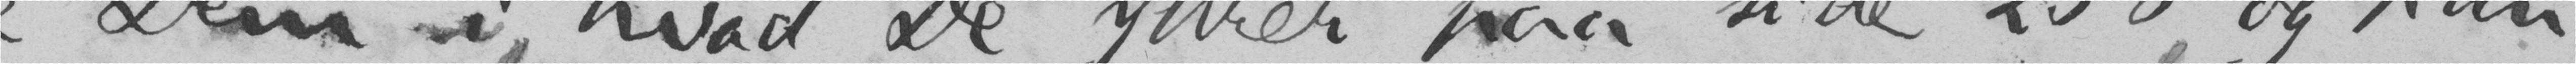Dem i, hvad De yttrer paa side 258, og kan
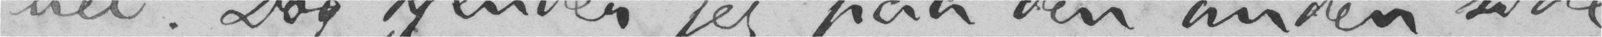uel. Dog kjender jeg paa den anden side
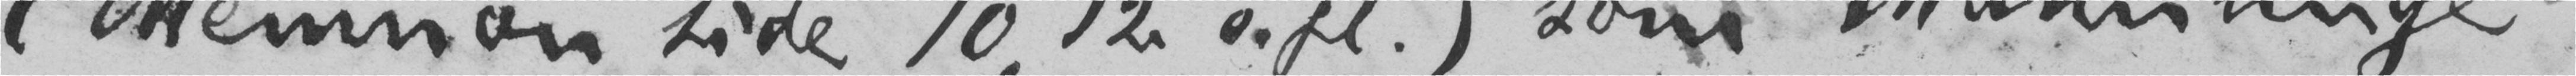(Memnon side 10, 12 o.fl.) som «Männlinge»
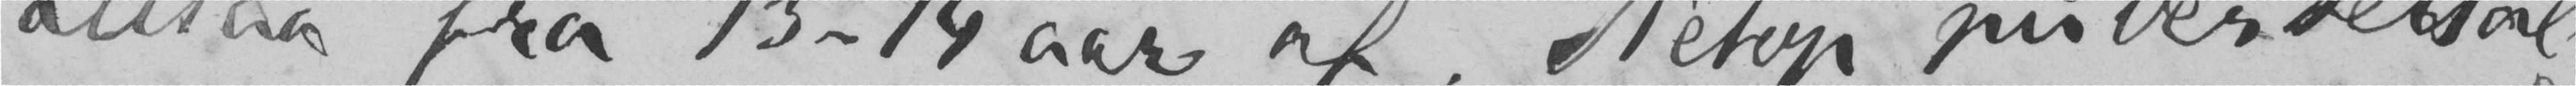altsaa fra 13-14 aar af. Netop pubertsal-
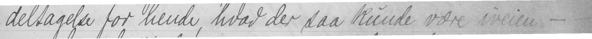deltagelse for hende, hvad der saa kunde være iveien - -
